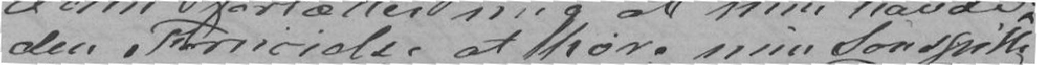den Fornøielse at høre min Søn spille
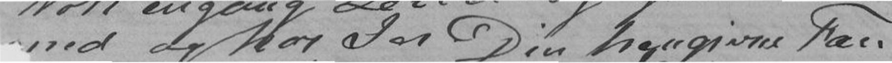ed og hos Jer. Din hengivne Faer
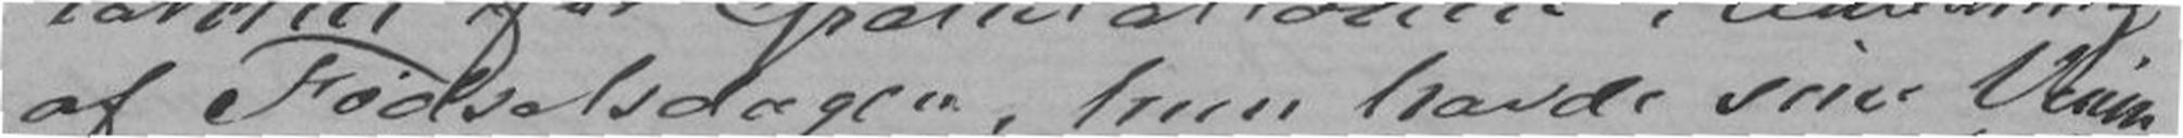af Fødseldagen, hun havde sine Venin-
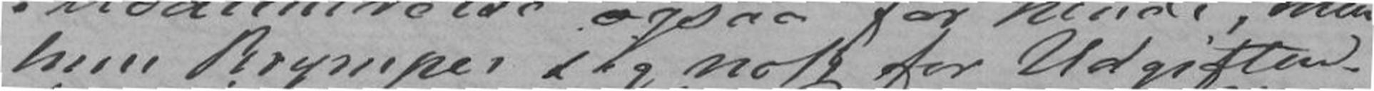hun krymper sig nok for Udgiften.
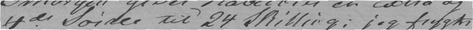4de Soiree til 24 Shilling; jeg frygter
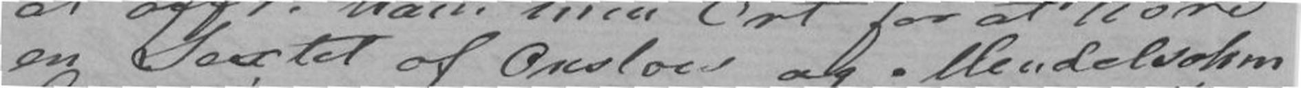en Sextet af Onslow og Mendelsohn
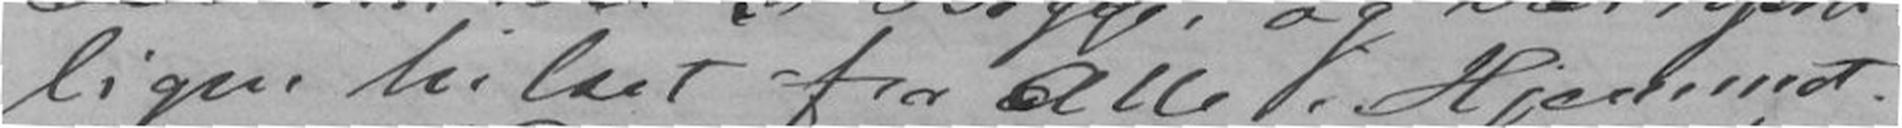ligen hilset fra Alle i Hjemmet.
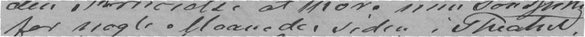for nogle Maaneder siden i Theatret,
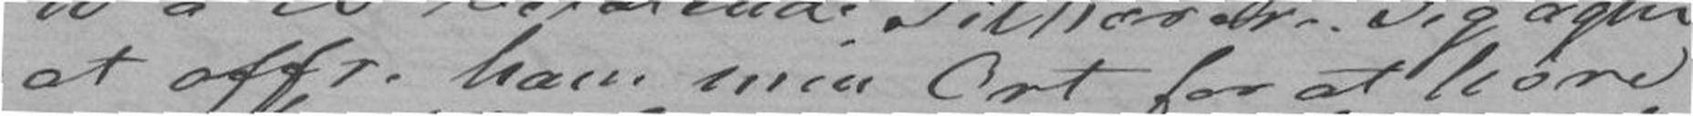at offre ham min Ort for at høre
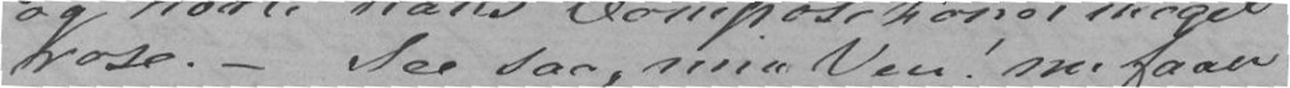rose. - See saa min Ven! nu faaer
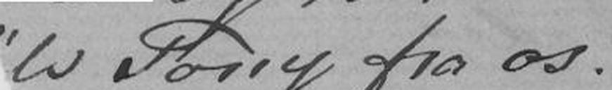ls Tony fra os.
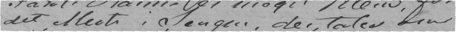det Meste i Sengen, der tales om
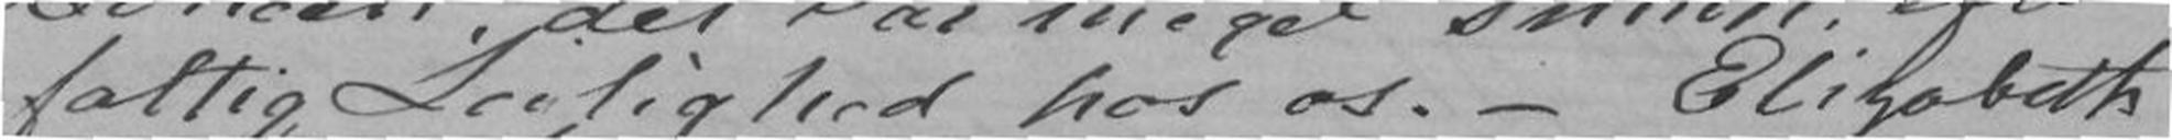fattig Leilighed hos os. - Elizabeth
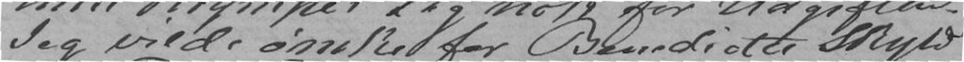Jeg vilde ønske for Benedictes Skyld
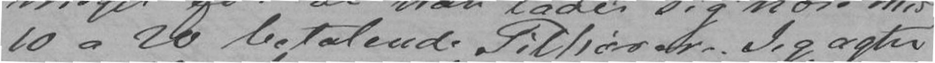10 a 20 betalende Tilhørere. Jeg agter
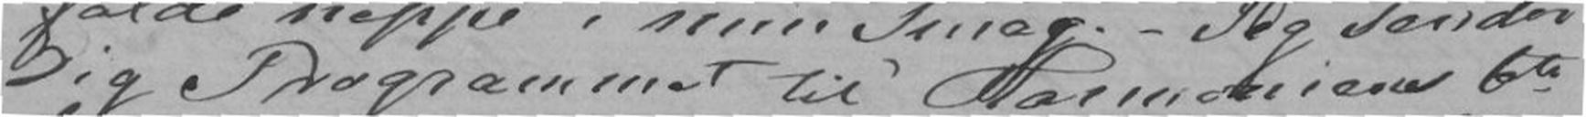Dig Programmet til Harmonien 6te
